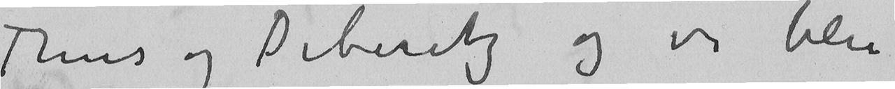Thiis og Deberitz og vi blev
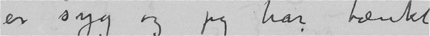er syg og jeg har tænkt
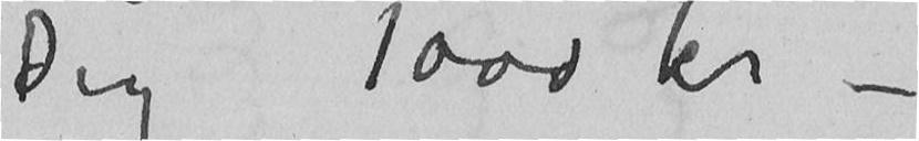Dig 1000 kr –
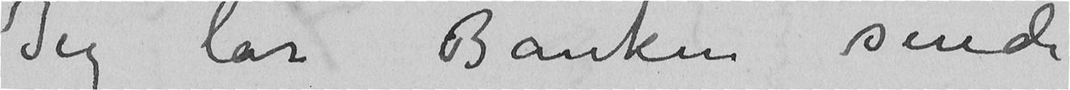Jeg lar Banken sende
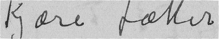Kjære fætter
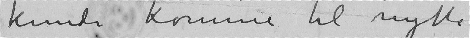kunde komme til nytte
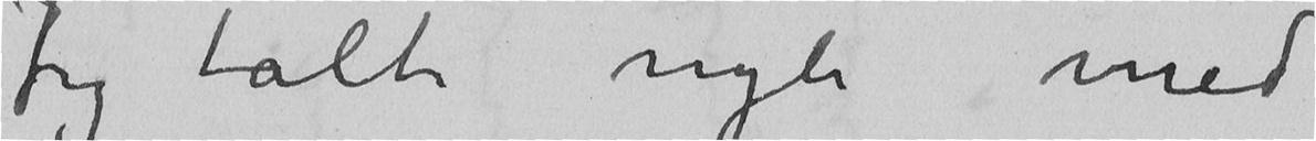Jeg talte nylig med
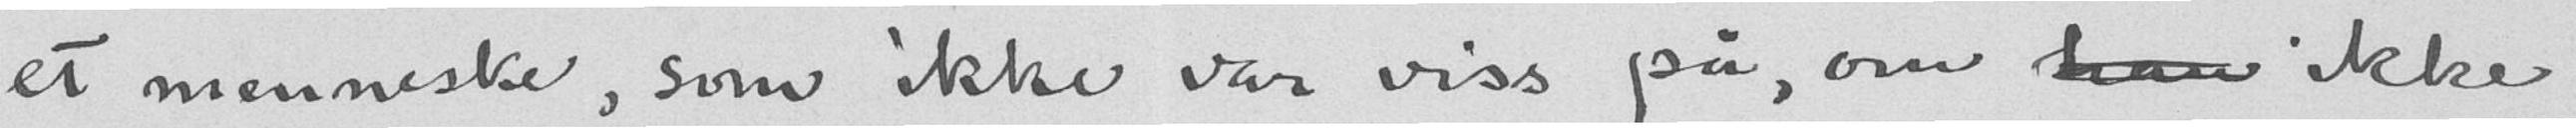et menneske, som ikke var viss på, om han ikke
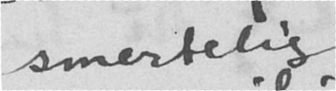smertelig
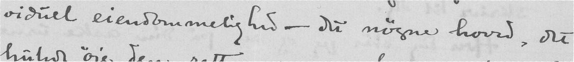viduel eiendommelighed - det nøgne hoved, det
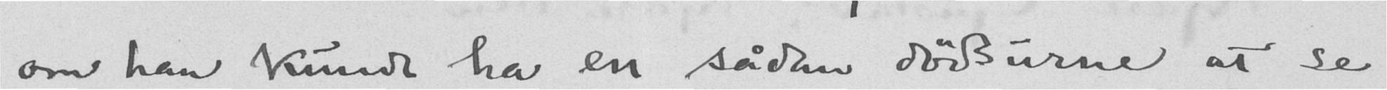om han kunde ha en sådan dødsurne at se
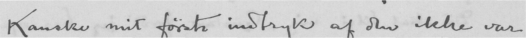Kanske mit første indtryk af den ikke var
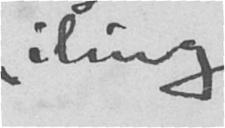iling.
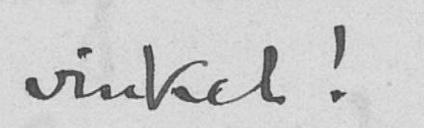vinkel!
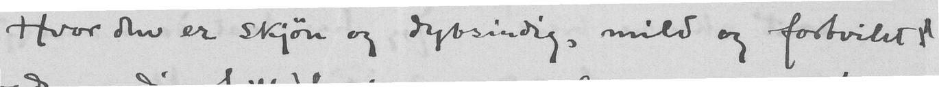Hvor den er skjøn og dybsindig, mild og fortvilet
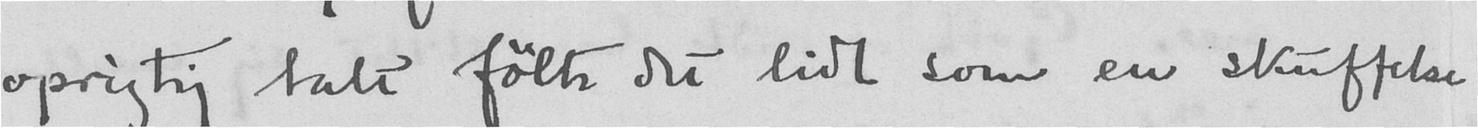oprigtig talt følte det lidt som en skuffelse
